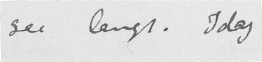saa langt. Idag
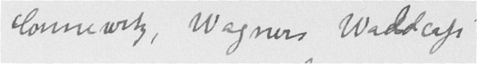Connewitz, Wagners Waldcafé
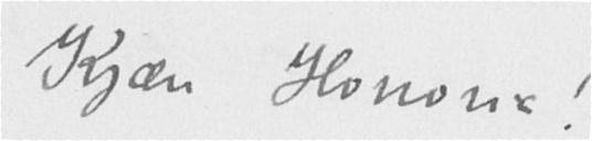Kjære Honoria!
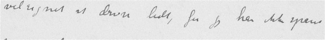velsignet at drove lidt, for jeg har ikke spire
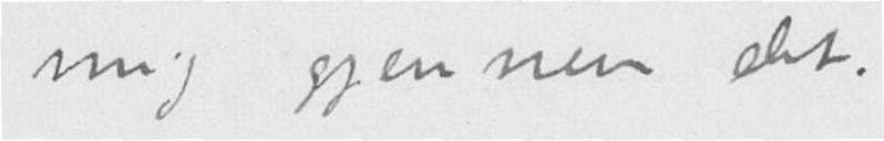mig gjennem det.
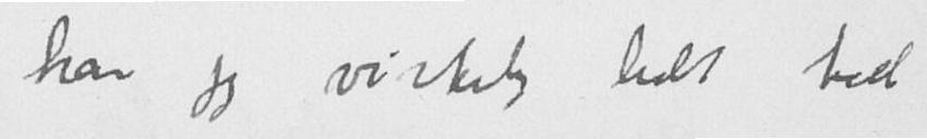har jeg virkelig lidt tid
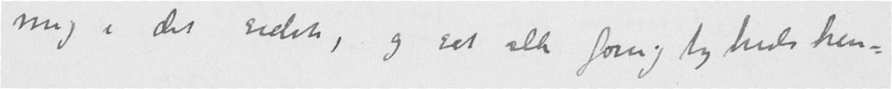mig i det sidste, og sat alle forsigtighedshen-
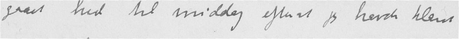gaaet hid til middag efterat jeg havde klart
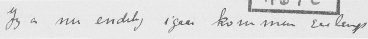Jeg er nu endelig igaar kommen saalangt
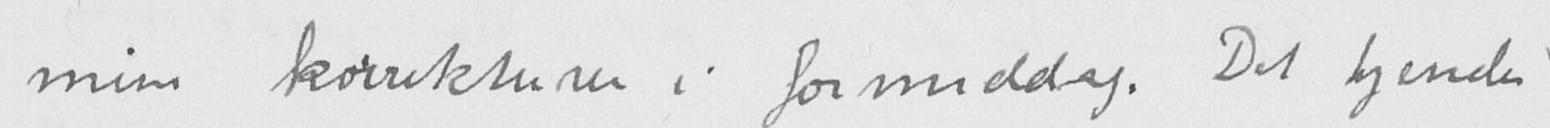mine korrekturer i formiddag. Det kjendes
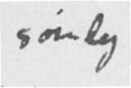søndag
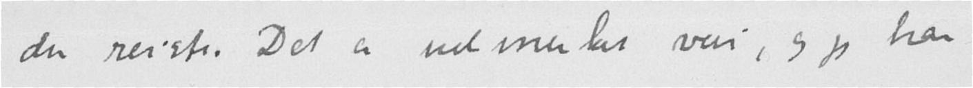du reiste. Det er udmerket veir, og jeg har
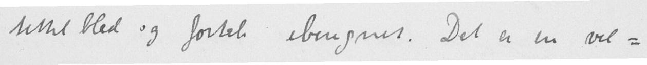tittelblad og fortale iberegnet. Det er en vel-
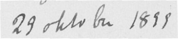29 oktober 1899
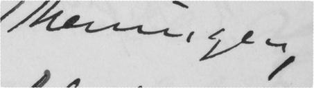Meningen,
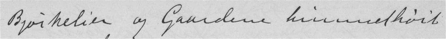Bjørkelien og Gaardene himmelhøit
